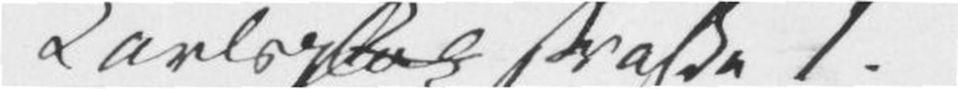Karlsplatz strasse 1.
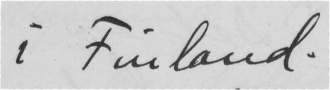i Finland.
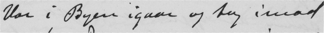Var i Byen igaar og tog imod
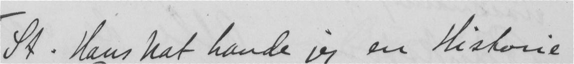St. Hans Nat havde jeg en Historie
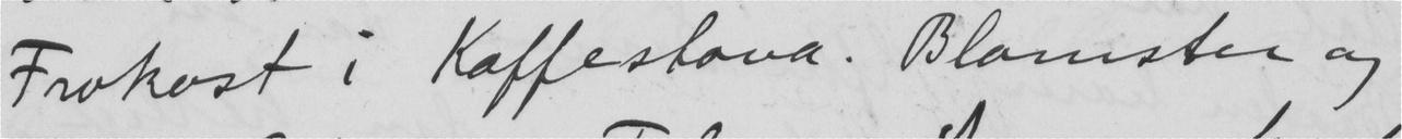Frokost i Kaffestova. Blomster og
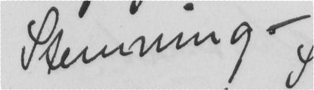Stemning.-
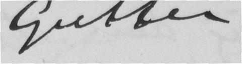Gutter
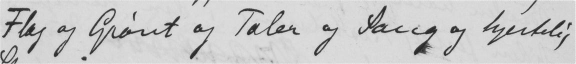Flag og Grønt og Taler og Sang og hjertelig
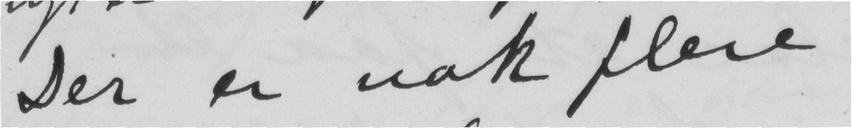Der er nok flere
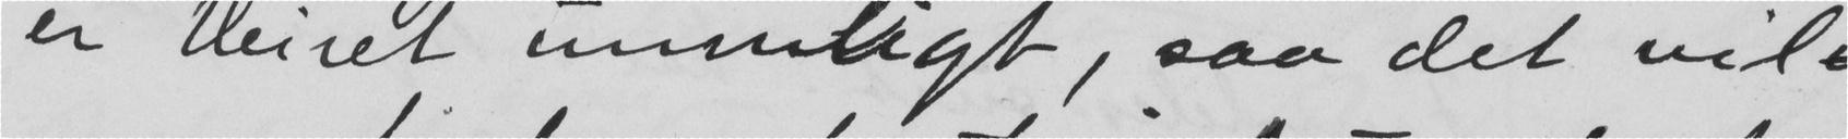er Veiret umuligt, saa det vil
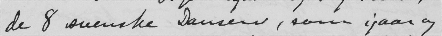de 8 svenske Dansere, som igaar og
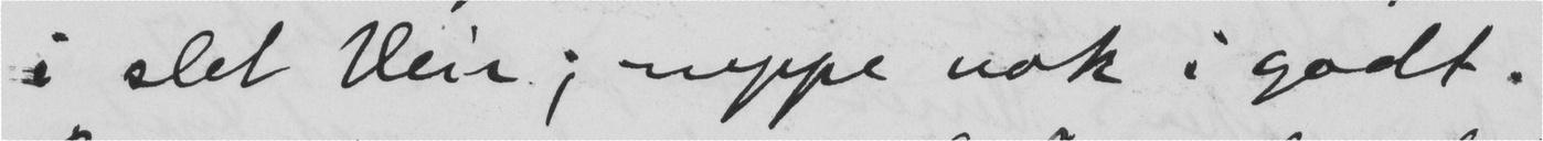i slet Veir; neppe nok i godt.
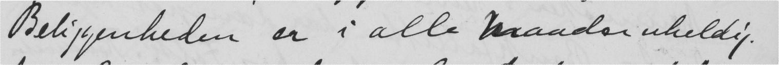Beliggenheden er i alle Maader uheldig.
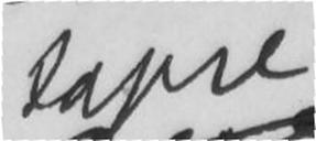tapre
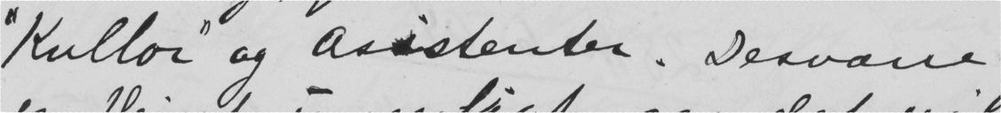"Kullor" og Asistenter. Desværre
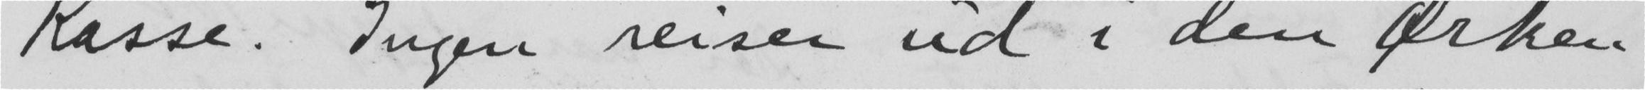Kasse. Ingen reiser ud i den Ørken
